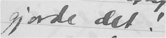gjorde det!
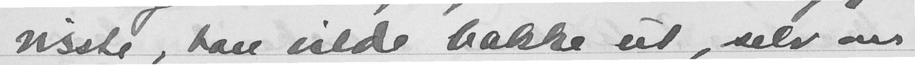visste, han vilde bakke ut, selv om
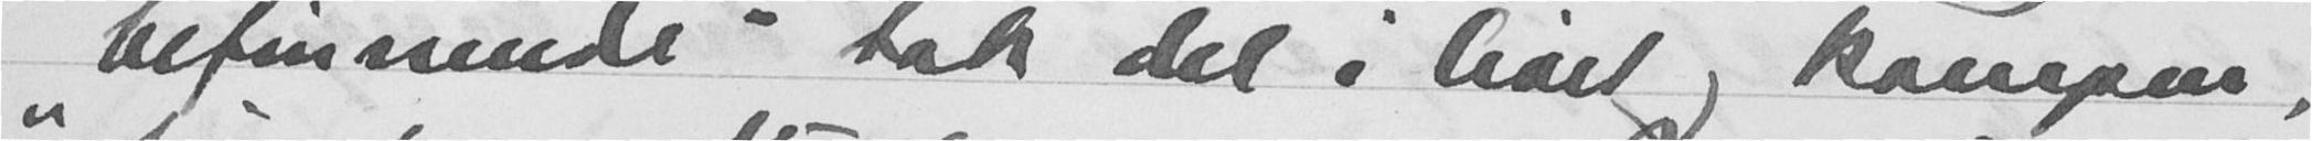"befinnende" tok del i livet og kampen,
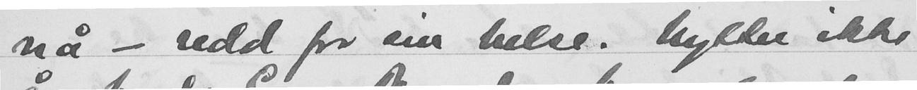nå - redd for sin helse. Nytter ikke
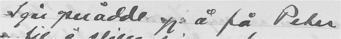Igår opnådde jeg å få Peter
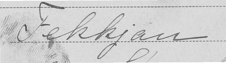Fekkjan
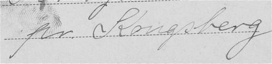for Kongsberg
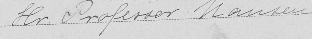Hr. Professor Nansen
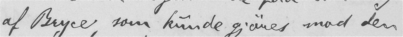af Bryce, som kunde gjøres mod den
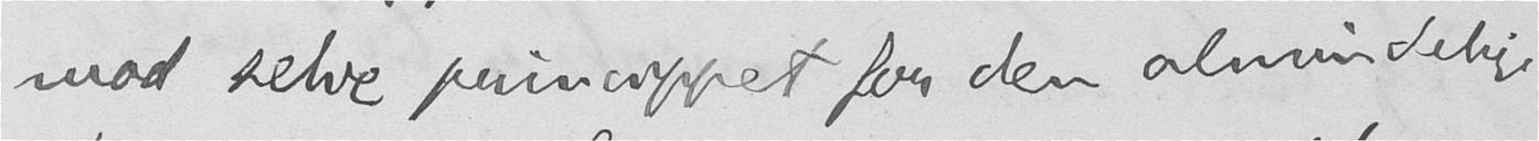mod selve princippet for den almindelige
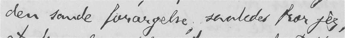den sande forargelse, saaledes tror jeg,
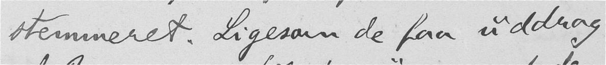stemmeret. Ligesom de faa uddrag
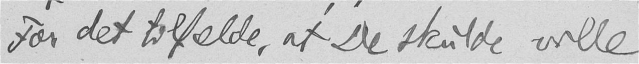For det tilfælde, at De skulde ville
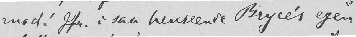mod! Jfr. i saa henseende Bryce's egen
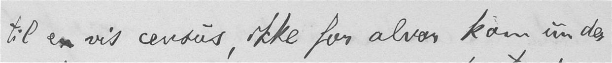til en vis census, ikke for alvor kom under
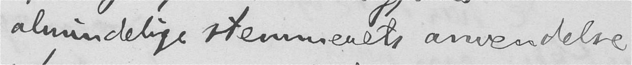almindelige stemmerets anvendelse
